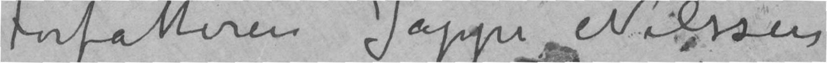Forfatteren Jappe Nilssen
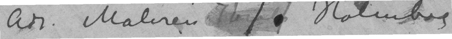Adr. Maleren ThorolfT. Holmboe
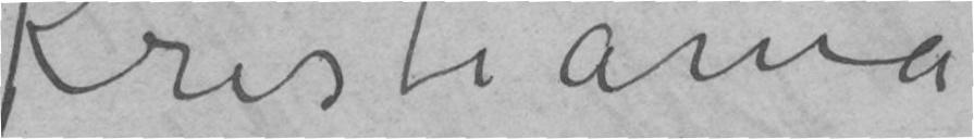Kristiania
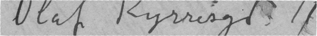Olaf Kyrresgd 11
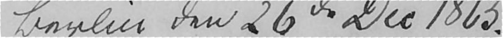Berlin den 26de Dec 1863.
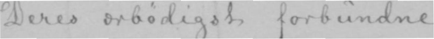Deres ærbødigst forbundne
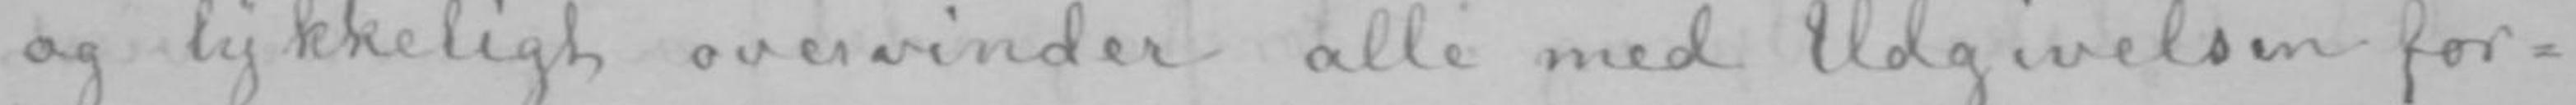og lykkeligt overvinder alle med Udgivelsen for-
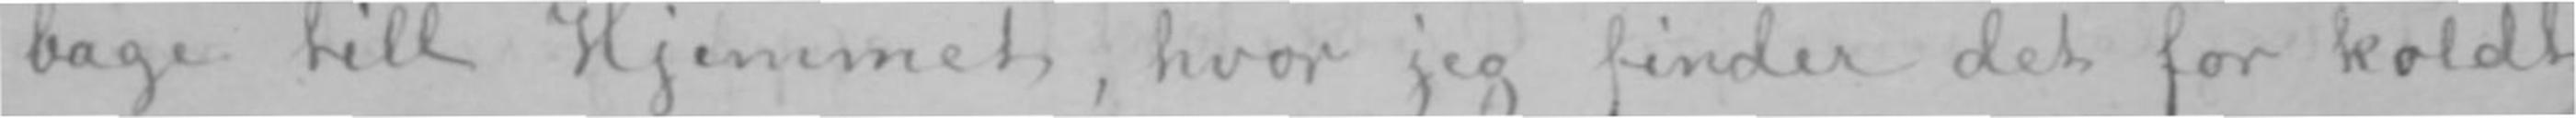bage till Hjemmet, hvor jeg finder det for koldt
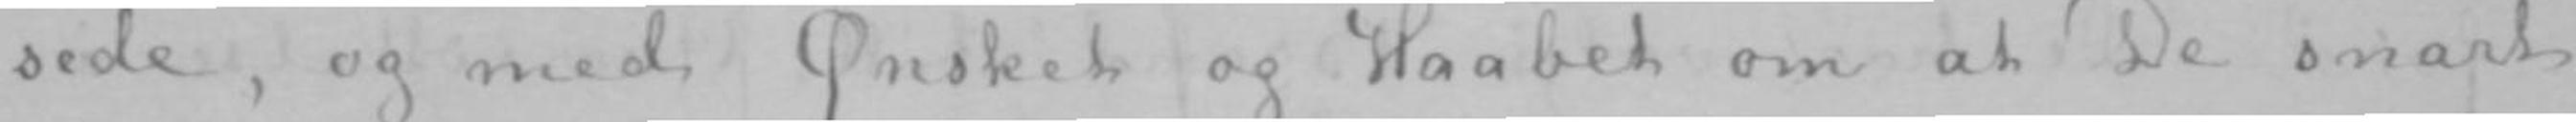sede, og med Ønsket og Haabet om at De snart
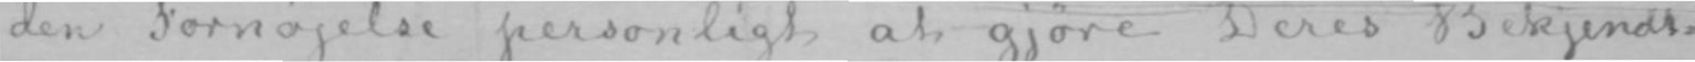den Fornøjelse personligt at gjøre Deres Bekjendt-
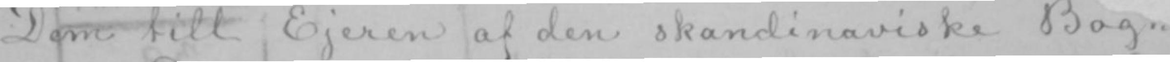Dem till Ejeren af den skandinaviske Bog
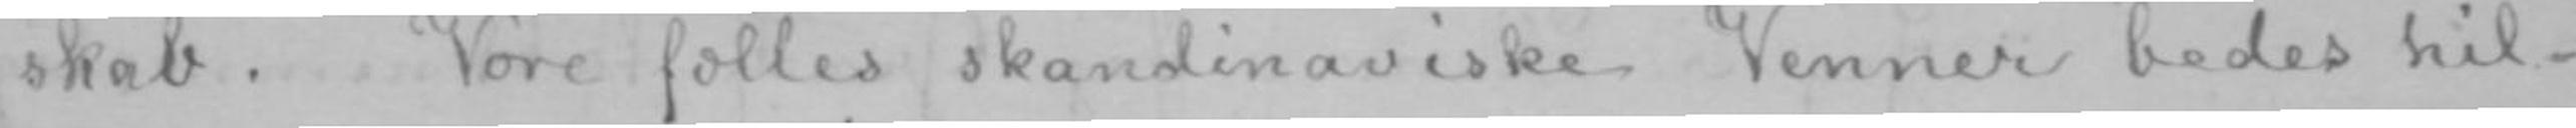skab. Vore fælles skandinaviske Venner bedes hil-
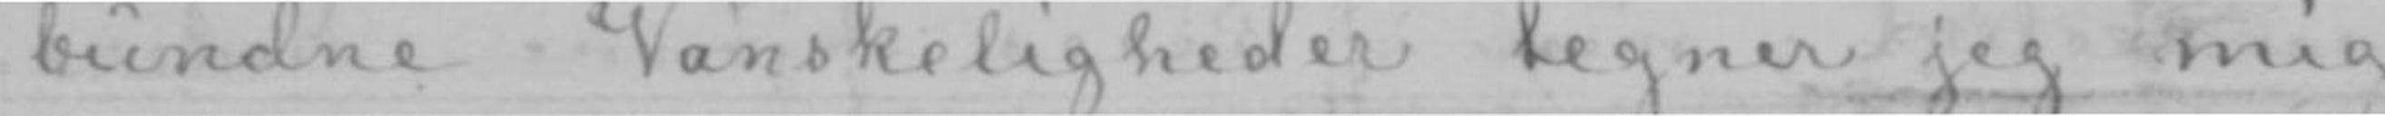bundne Vanskeligheder tegner jeg mig
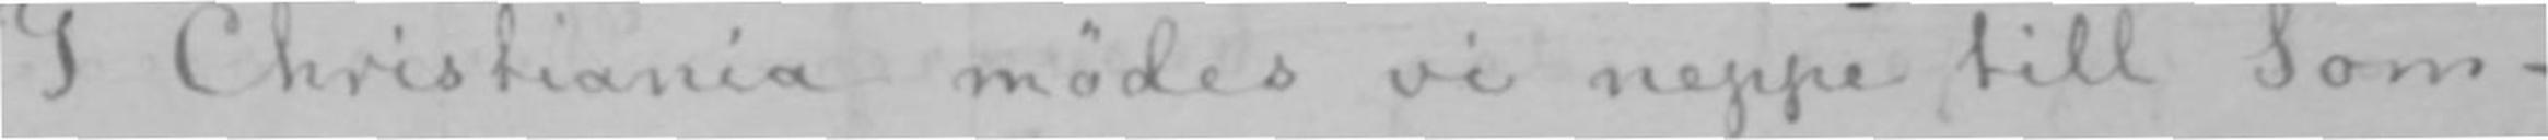I Christiania mødes vi neppe till Som-
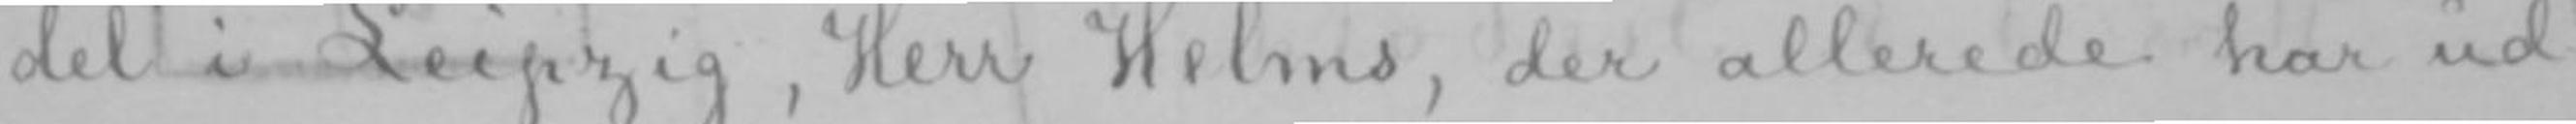del i Leipzig, Herr Helms, der allerede har ud-
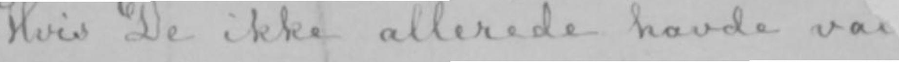Hvis De ikke allerede havde va
